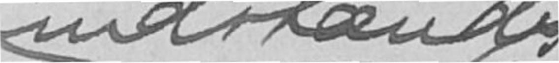indstændig
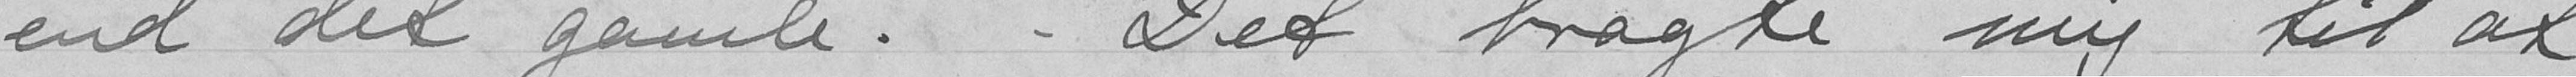en det gamle. Det bragte mig til at
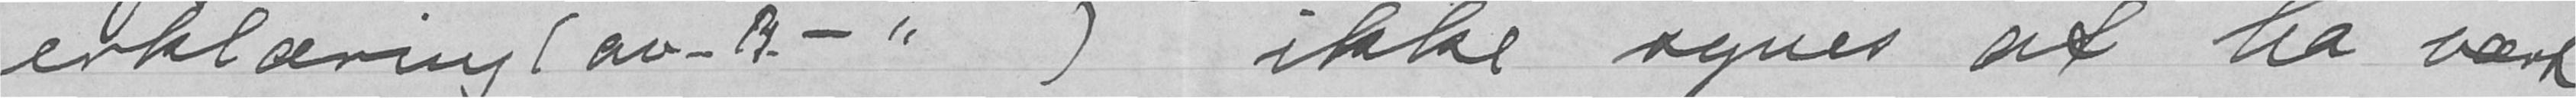erklæring (av - B. - " ) ikke synes at ha vært
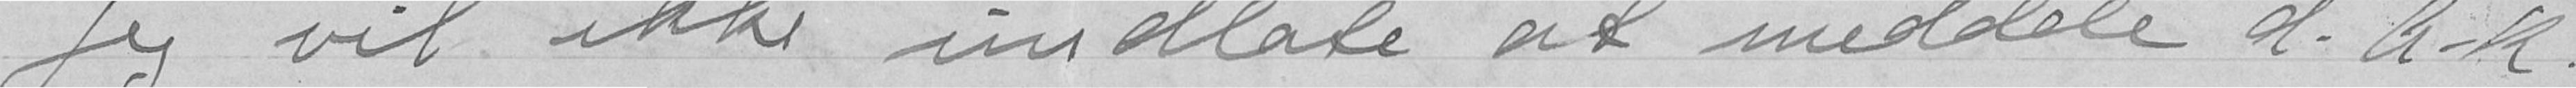Jeg vil ikke undlate at meddele H. A-K
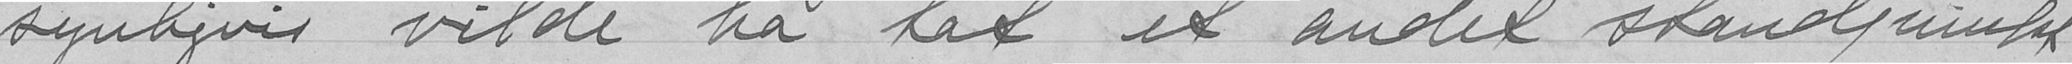synligvis vilde ha hat et andet standpunkt
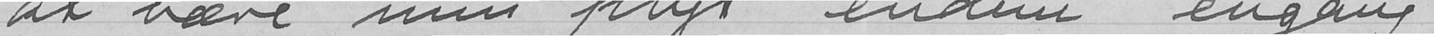at være min pligt endnu engang
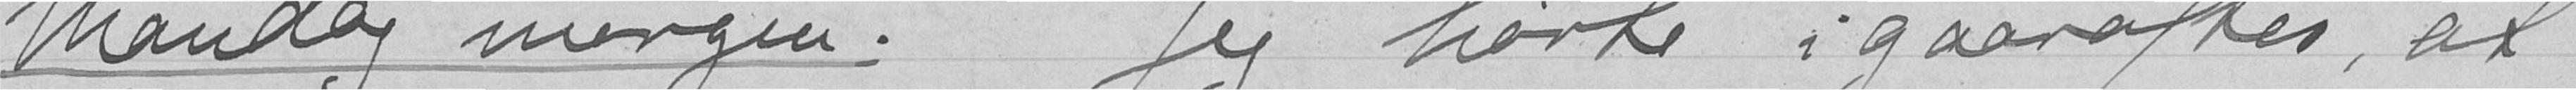Mandag morgen. Jeg hørte i gaaraftes, at
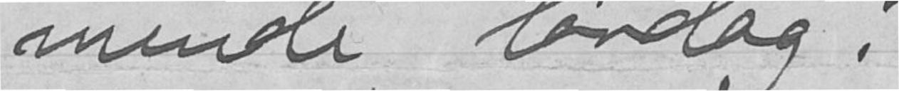mende lørdag.
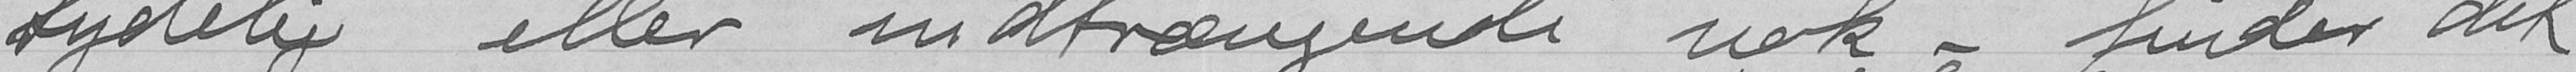tydelig eller indtrængende nok - finder det
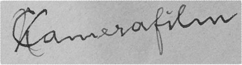Kamerafilm
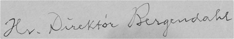Hr. Direktør Bergendahl
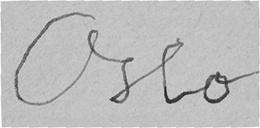Oslo
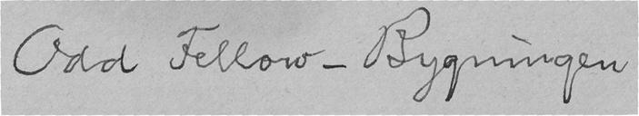Odd Fellow-Bygningen
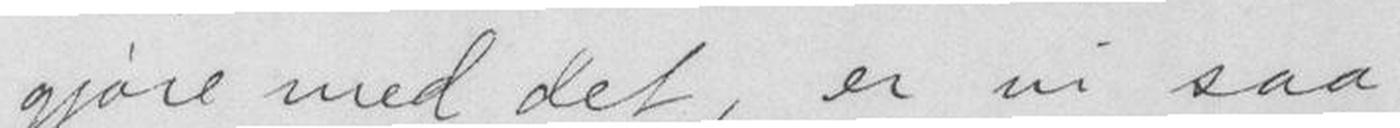gjøre med det, er vi saa
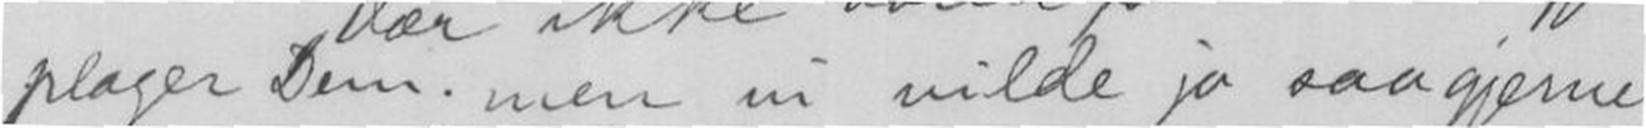plager Dem, men vi vilde jo saa gjerne
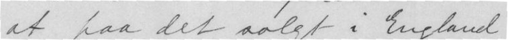at faa det solgt i England,
